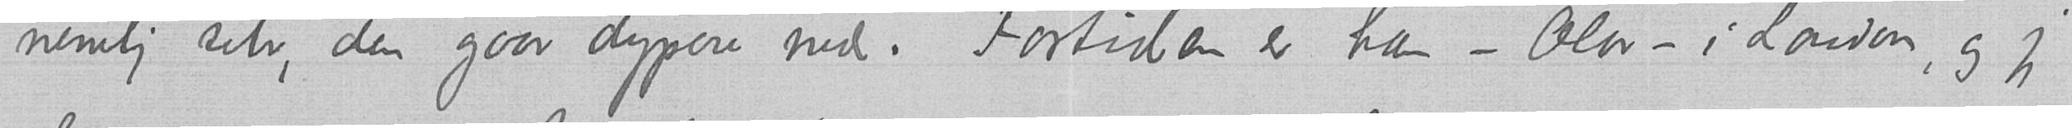nemlig selv, den gaar dypere ned. Fortiden er han – Olav – i London, og jeg
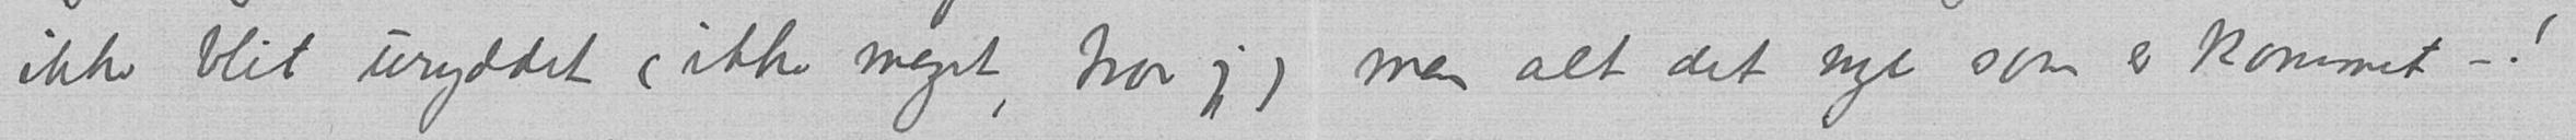ikke blit uryddet (ikke meget, tror jeg) men alt det nye som er kommet –
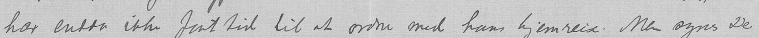har endda ikke faat tid til at ordne med hans hjemreise. Men synes De
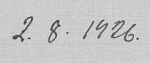2.8.1926.
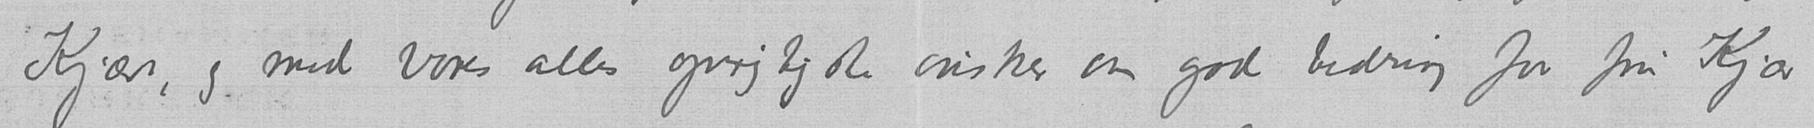Kjær, og med vores alles oprigtigste ønsker om god bedring for fru Kjær
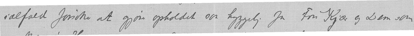ialfald forsøke at gjøre opholdet saa hyggelig for Fru Kjær og Dem som
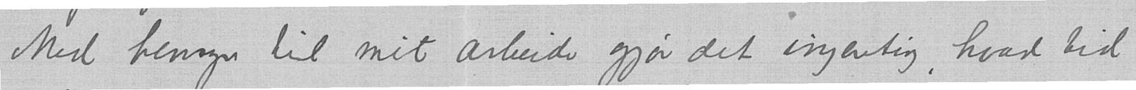Med hensyn til mit arbeide gjør det ingentig, hvad tid
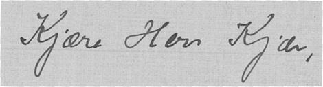Kjære Herr Kjær,
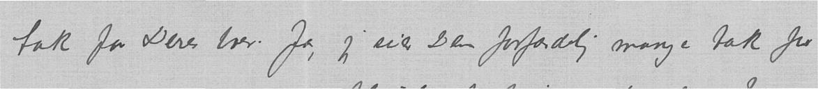tak for Deres brev. Ja, jeg sier Dem forfærdelig mange tak for
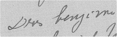– og altsaa paa gjensyn
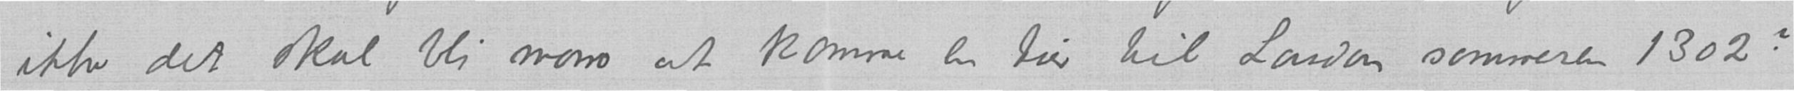ikke det skal bli morro at komme en tur til London sommeren 1302?
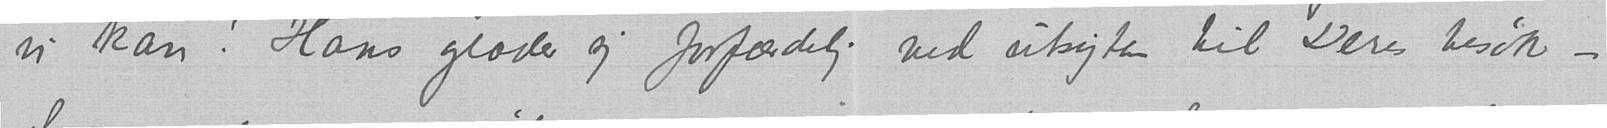vi kan. Hans glæder sig forfærdelig ved utsigten til Deres besøk –
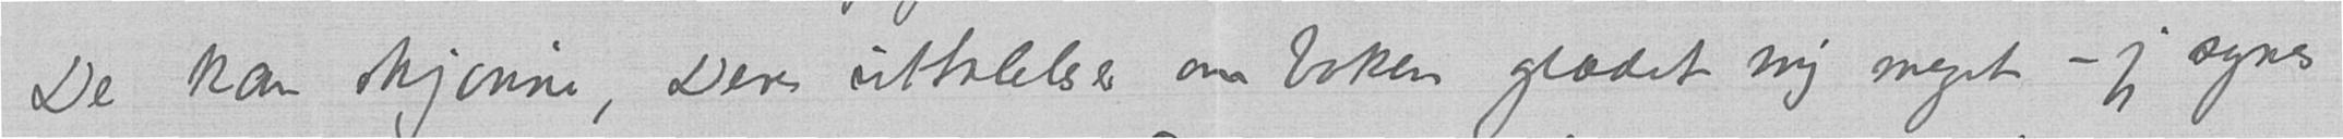De kan skjønne, Deres uttalelser om boken glædet mig meget – jeg syns
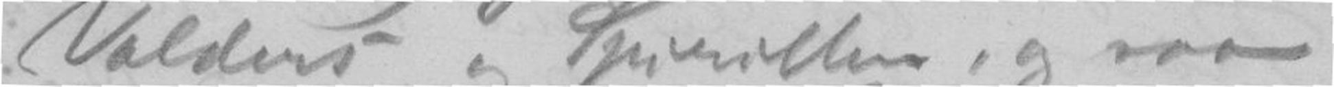Valders og Spirillen, og saa
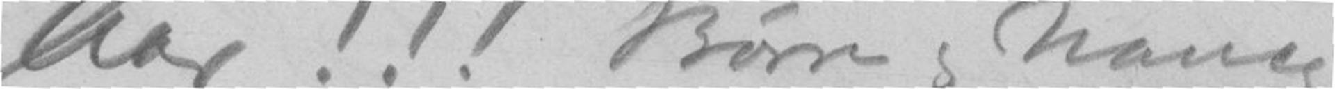Aar!!! Børre og Nancy
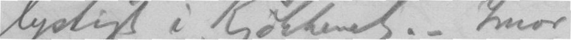lystigt i Kjøkkenet. - Imor-
